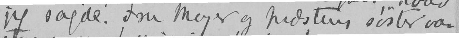jeg sagde. Fru Meyer og præstens søster var
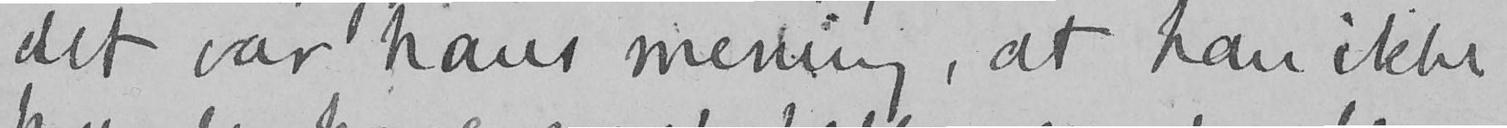det var hans mening, at han ikke
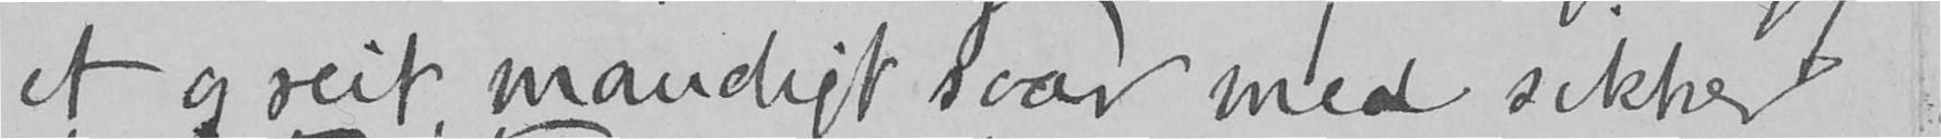et greit, mandigt svar med sikker
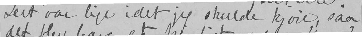Det var lige idet jeg skulde kjøre, saa
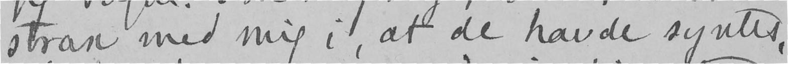strax med mig i, at de havde syntes,
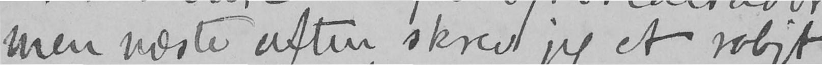men næste aften skrev jeg et roligt
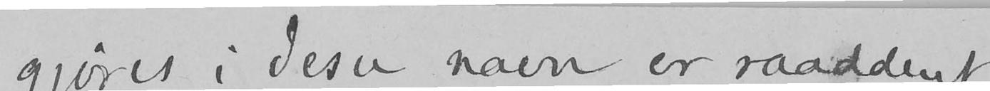gjøres i Jesu navn er raaddent
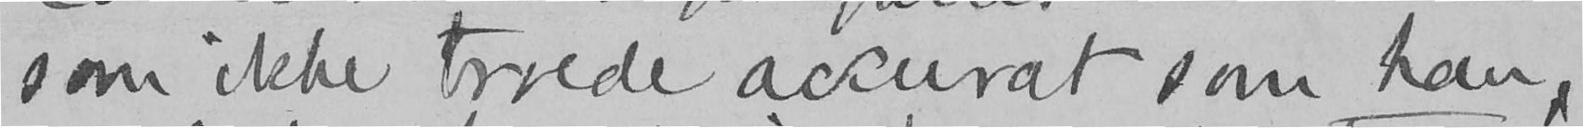som ikke troede accurat som han,
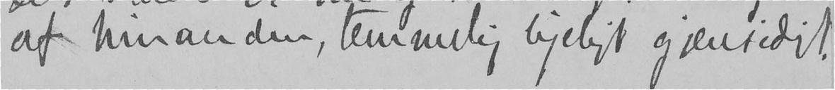af hinanden, temmelig ligeligt gjensidigt.
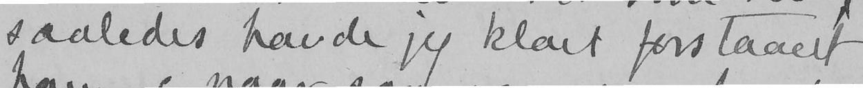saaledes havde jeg klart forstaaet
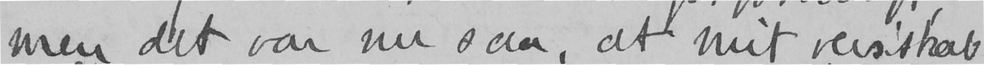men det var nu saa, at mit venskab
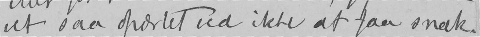vet saa opærtet ved ikke at faa snak-
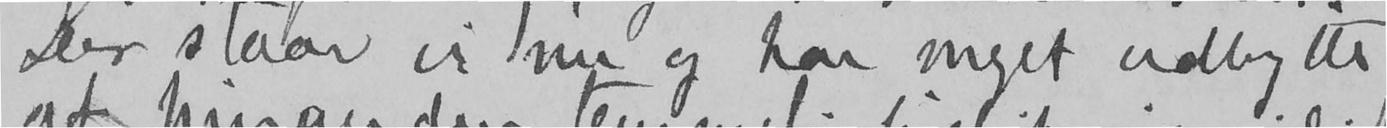Der staar vi nu og har meget udbytte
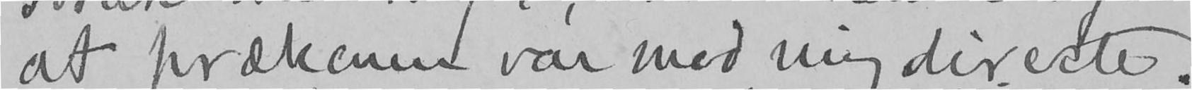at prækenen var mod mig directe.
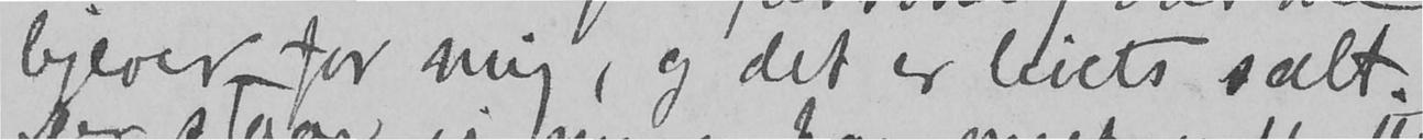ligeover for mig, og det er livets salt.
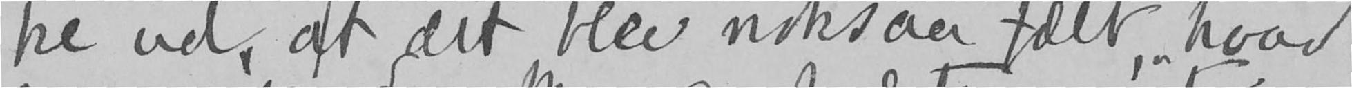ke ud, at det blev noksaa fælt, hvad
